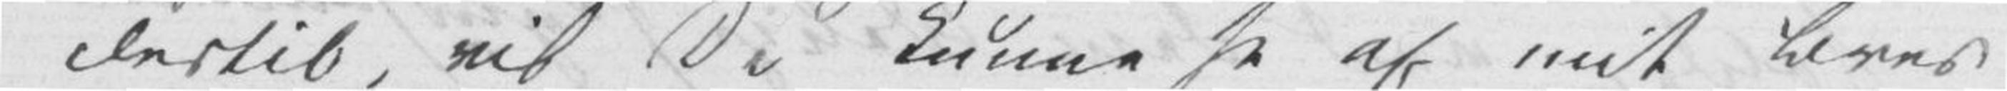dertil, vil De kunne se af mit Brev
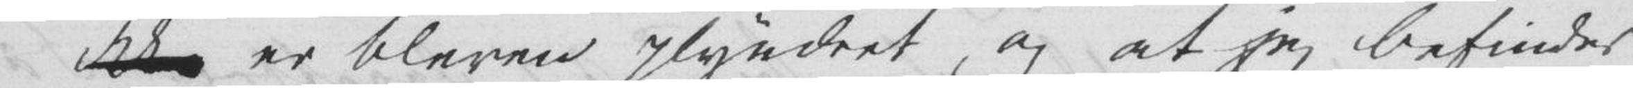ikke er bleven plyndret og at jeg befinder
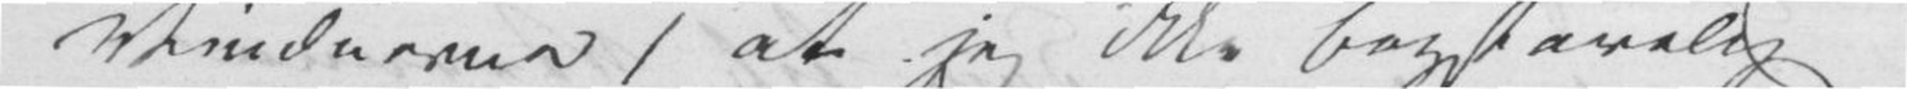Vinduerne) at jeg ikke bogstavelig
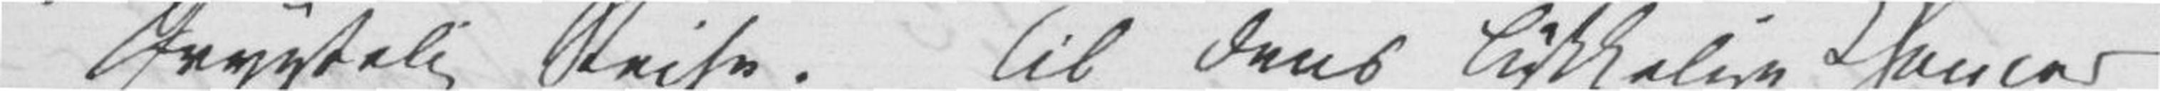frygtelig Reise. Til dens lykkelige Chancer
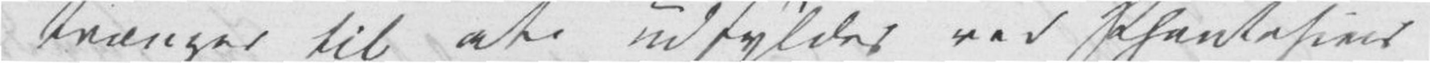trænger til at udfyldes ved Phantasiens
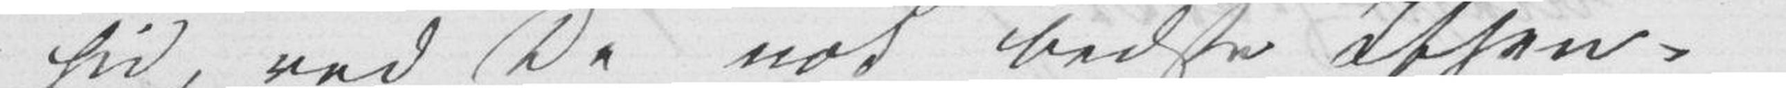hid, ved De nok bedste Ibsen,
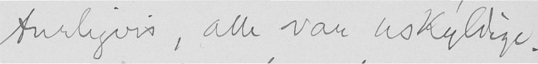turligvis, alle var uskyldige.
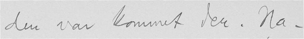den var kommet der. Na-
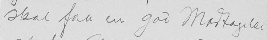skal faa en god Modtagelse
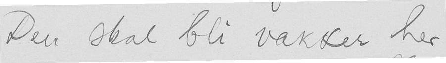Den skal bli vakker her
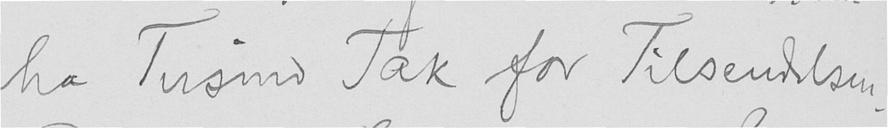ha Tusind Tak for Tilsendelsen.
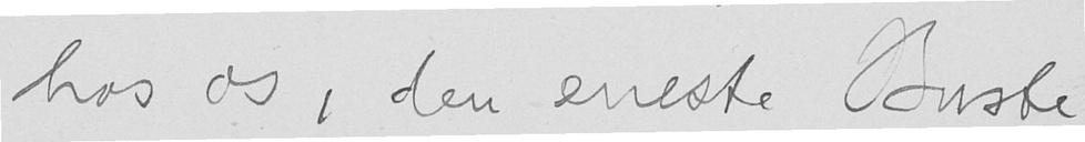hos os, den eneste Buste
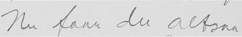Nu faar du altsaa
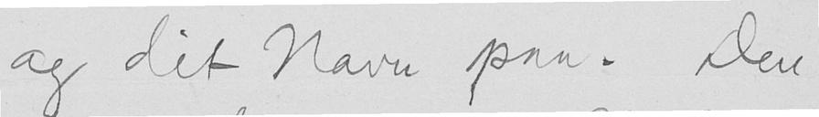og dit Navn paa. Den
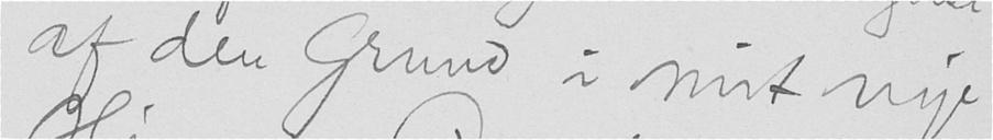af den Grund i mit nye
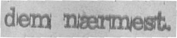dem nærmest.
